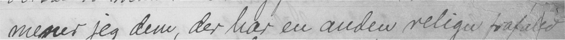mener jeg dem, der har en anden relign frafaled
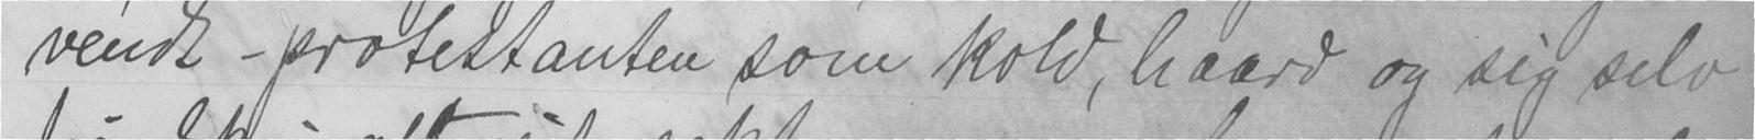vendt - protestanten som kold, haard og sig selv
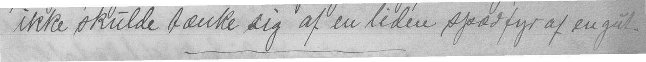ikke skulde tænke sig af en liden spæd fyr af en gut.
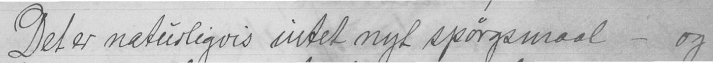Det er naturligvis intet nyt spørgsmaal - og
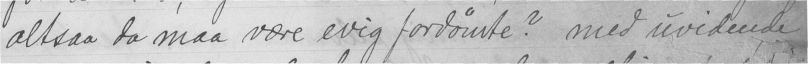altsaa da maa være evig fordømte? med uvidende
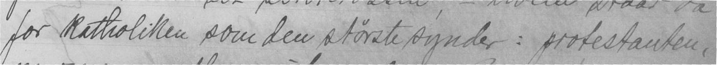for katholiken som den største synder: protestanten,
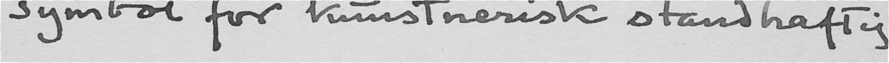symbol for kunstnerisk standhaftig-
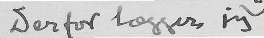Derfor lægger jeg
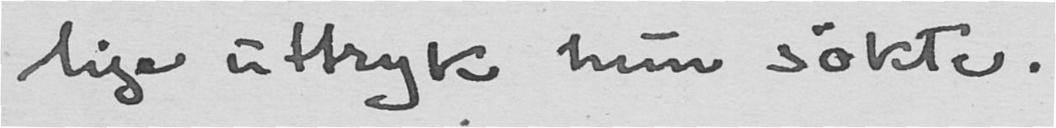lige uttryk hun søkte.
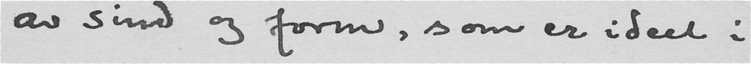av sind og form, som er ideel i
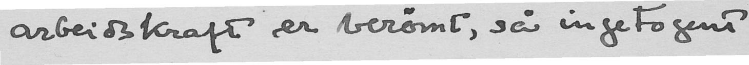arbeidskraft er berømt, så ingetogent
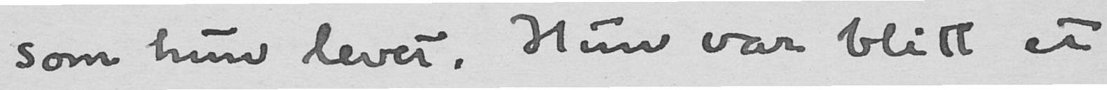som hun levet. Hun var blitt et
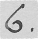6.
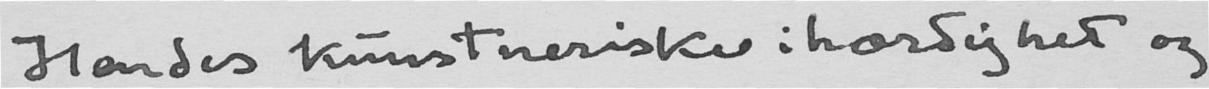Hændes kunstneriske ihærdighet og
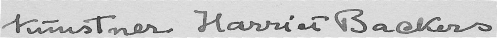kunstner Harriet Backers
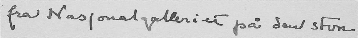fra Nasjonalgalleriet på den store
